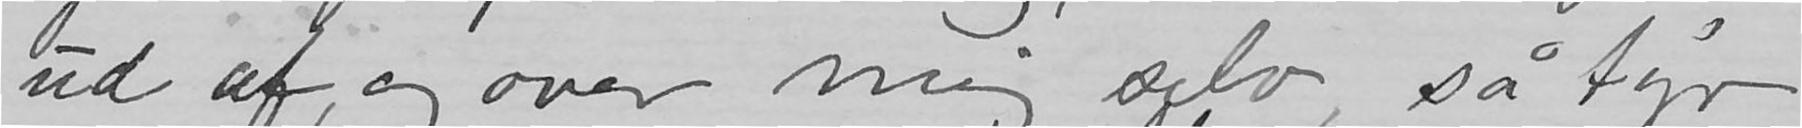ud af, og over mig selv, så tyr
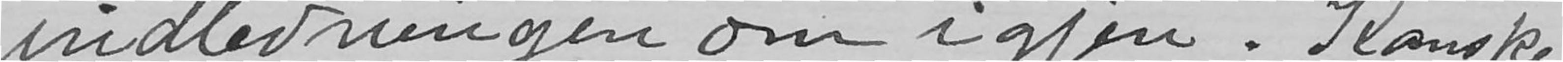indledningen om igjen. Kanske
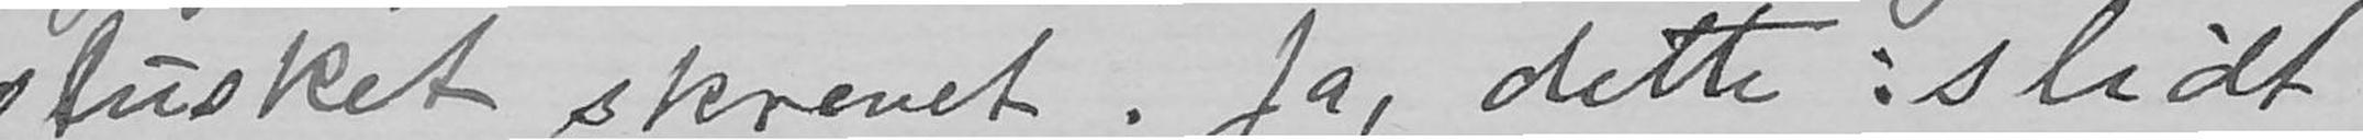slusket skrevet. Ja, dette: slidt
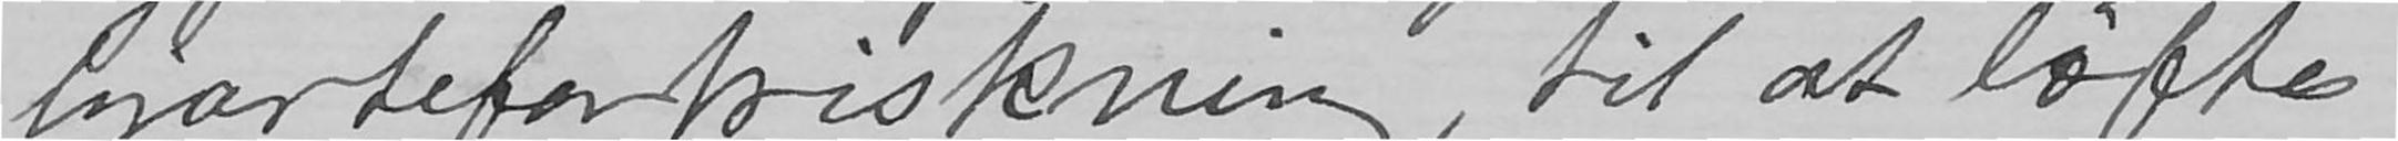hjærteforfriskning, til at løftes
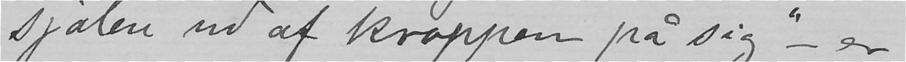sjælen ud af kroppen på sig"- er
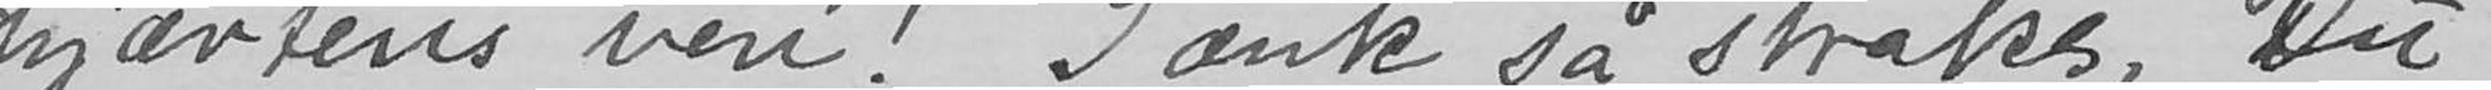hjærtens ven! Tænk så straks, Du
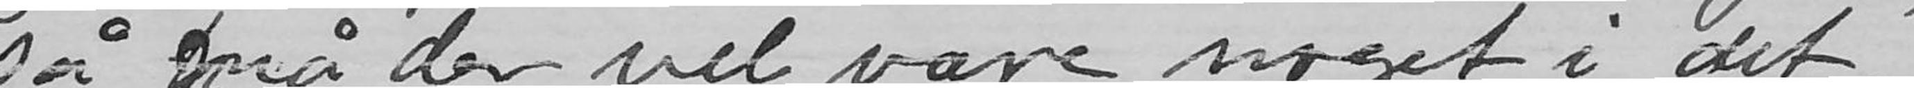så må der vel være noget i det.
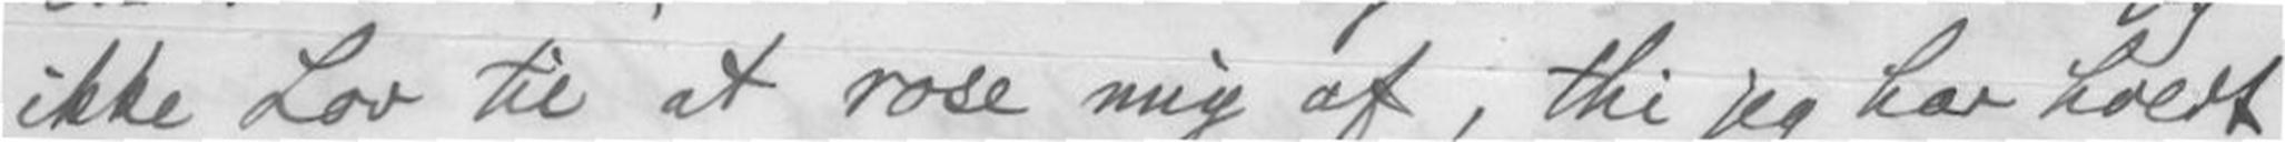ikke Lov til at rose mig af, thi jeg har holdt
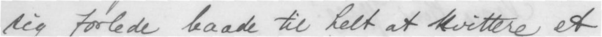sig forlede baade til helt at kvittere et
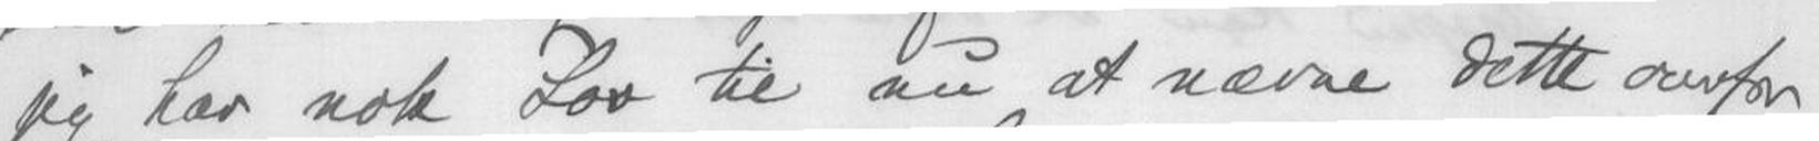jeg har nok Lov til nu at nævne dette overfor
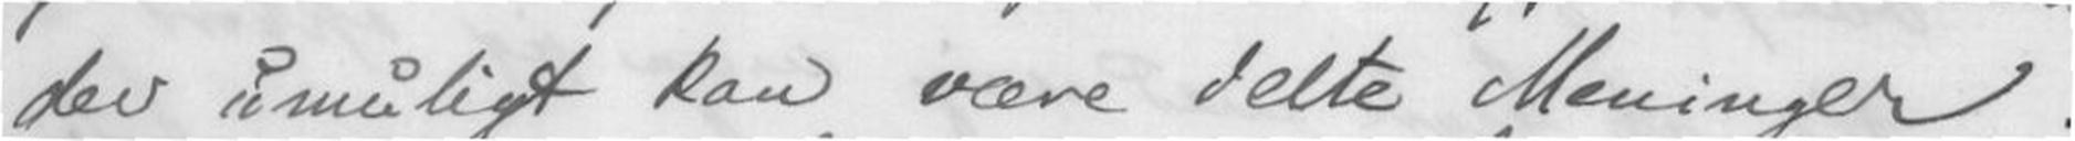det umuligt kan være delte Meninger.
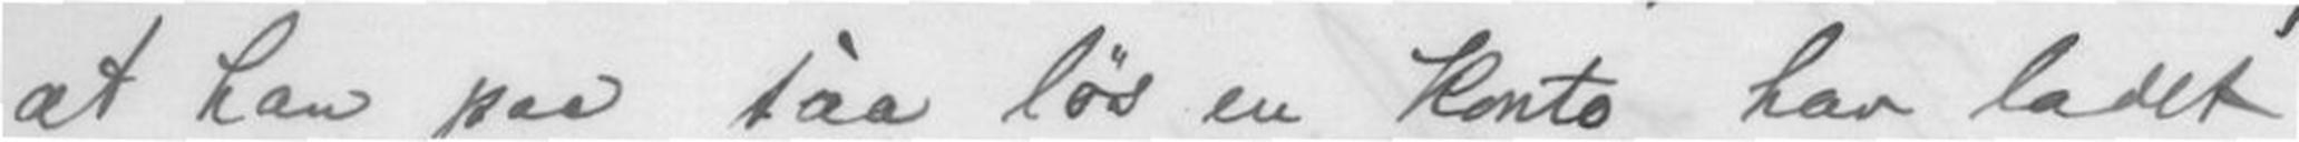at han paa saa løs en konto har ladet
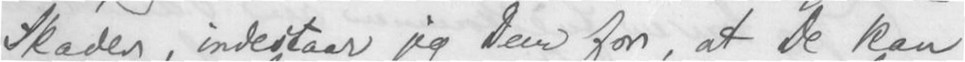Skader, indestaar jeg Dem for, at De kan
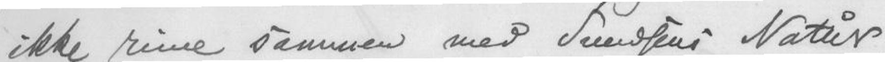ikke ri.. sammen med Svendsens Natur
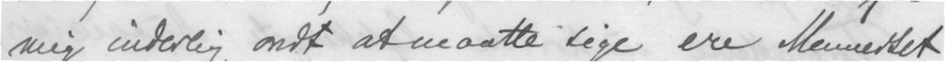mig inderlig ondt at maatte sige ere Mennesker
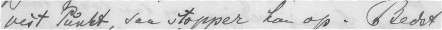vist Punkt, saa stopper han op. Bedst
